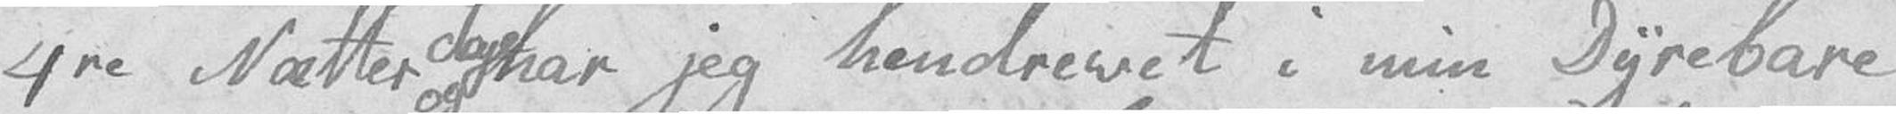4re Nætter og dage har jeg hendrevet i min Dyrebare
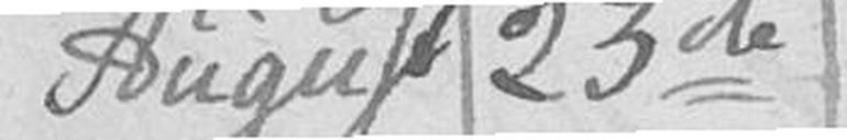August 23de
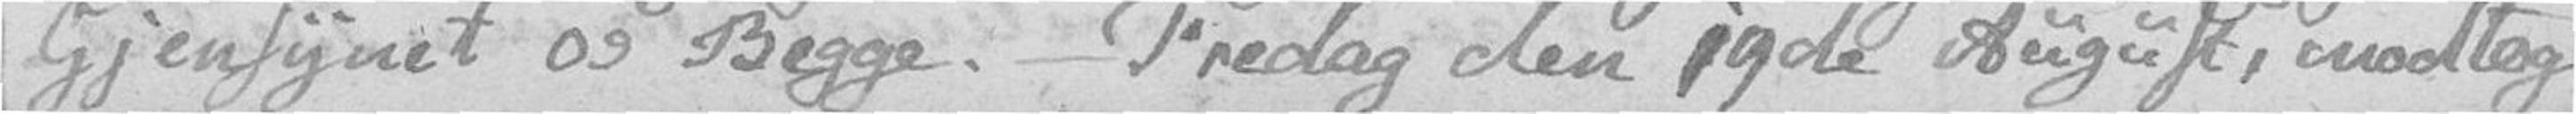Gjensynet os Begge. – Fredag den 19de August, modtog
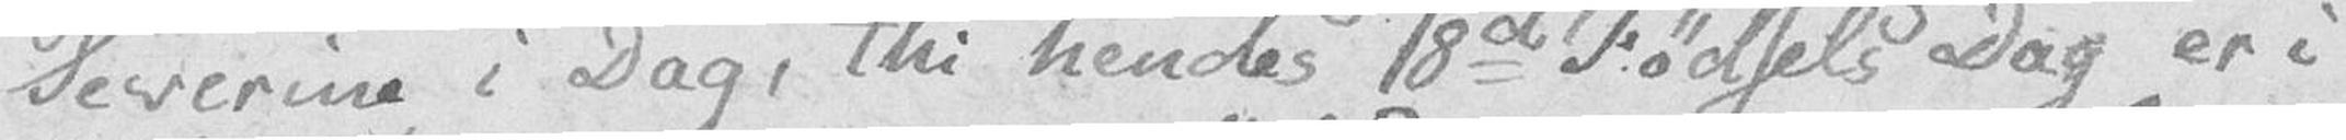Severine i Dag, thi hendes 18de Fødsels Dag er i
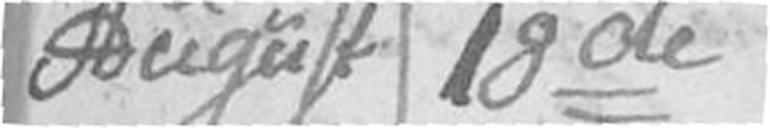August 18de
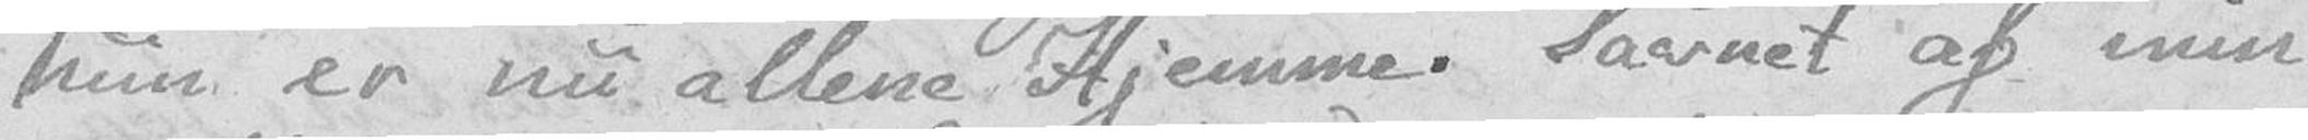hun er nu allene Hjemme. Savnet af min
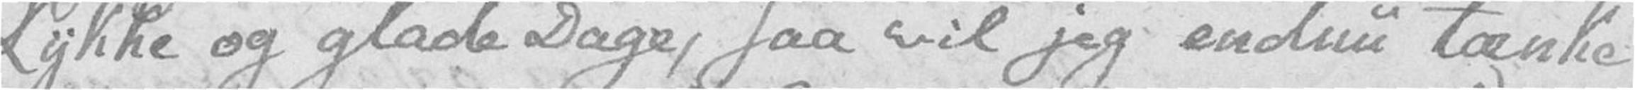Lykke og glade Dage, saa vil jeg endnu tænke
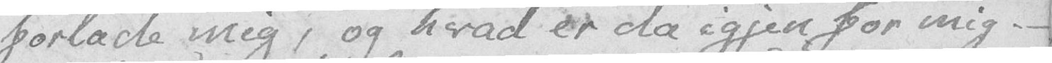forlade mig, og hvad er da igjen for mig. –
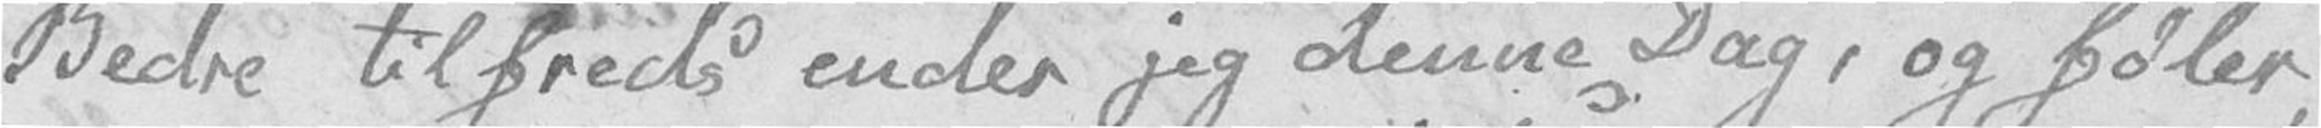Bedre tilfreds ender jeg denne Dag, og føler
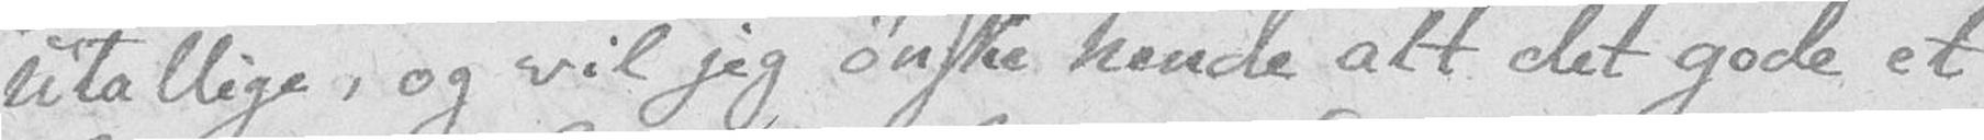Utallige, og vil jeg ønske hende alt det gode et
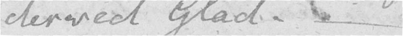derved Glad. –
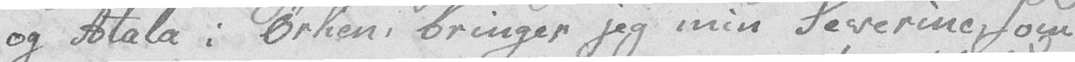og Atala i Ørken, bringer jeg min Severine, som
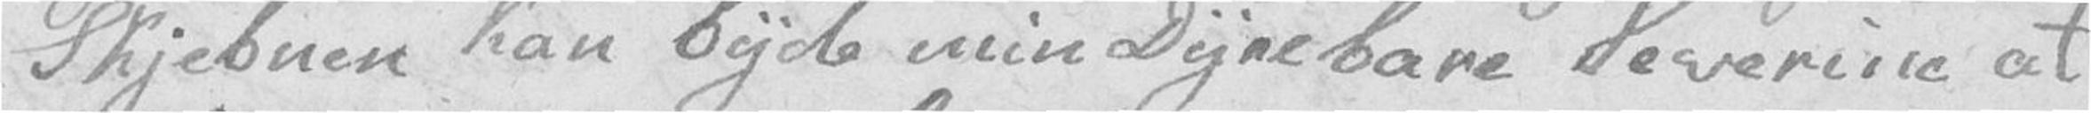Skjebnen kan byde min Dyrebare Severine at
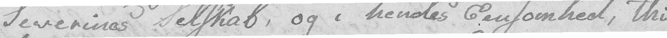Severines Selskab, og i hendes Eensomhed, thi
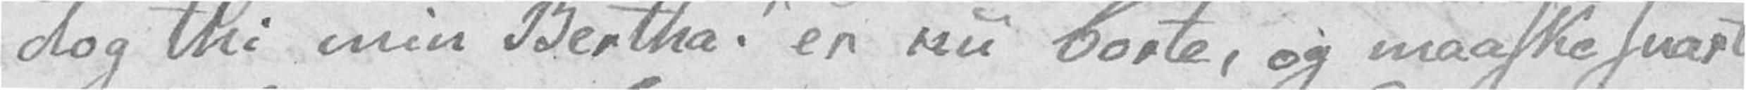dog thi min Bertha! er nu borte, og maaske snart
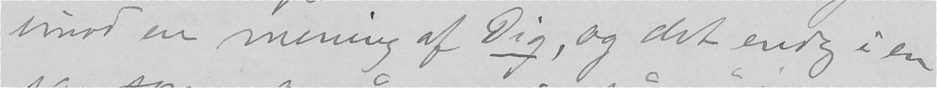imod en mening af Dig, og det endog i en
